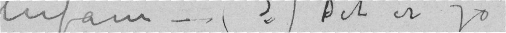infam – (?) Det er jo
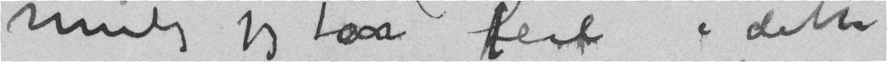mulig jeg tar feil i dette
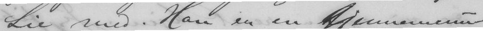Lie med. Han er en Gjennemmu-
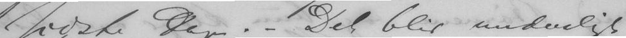sidste Dage. - Det blir underligt
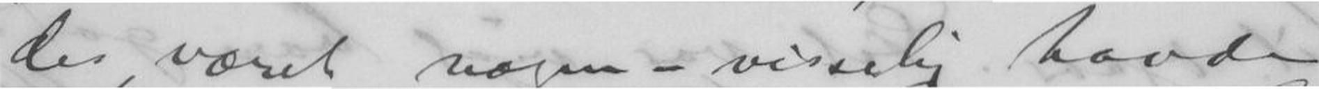de været nogen - visselig havde
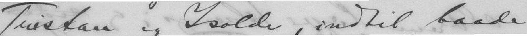Tristan og Isolde, indtil baade
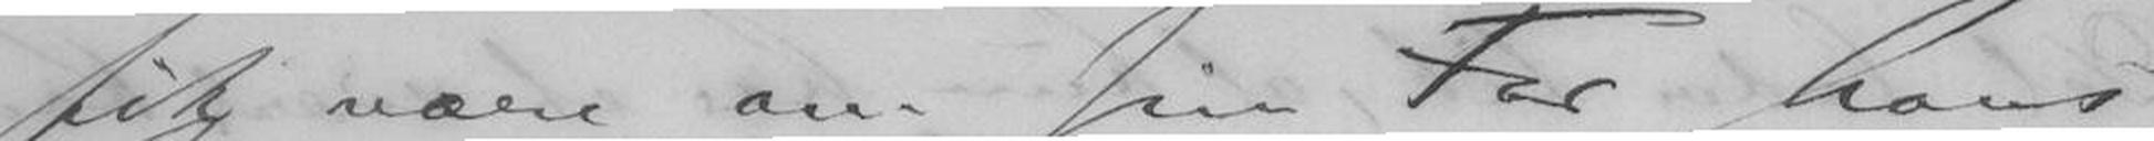fik være om sin Far hans
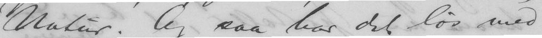Natur. Og saa bar det løs med
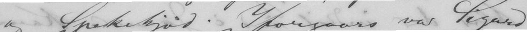og Spekekjød. Iforgaars var Sigurd
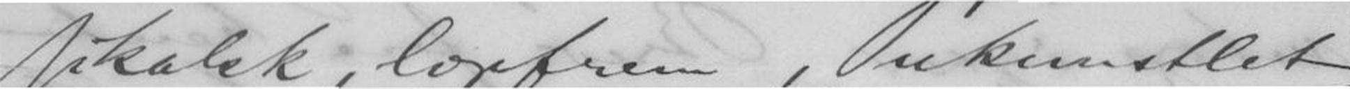sikalsk, ligefrem, ukunstlet
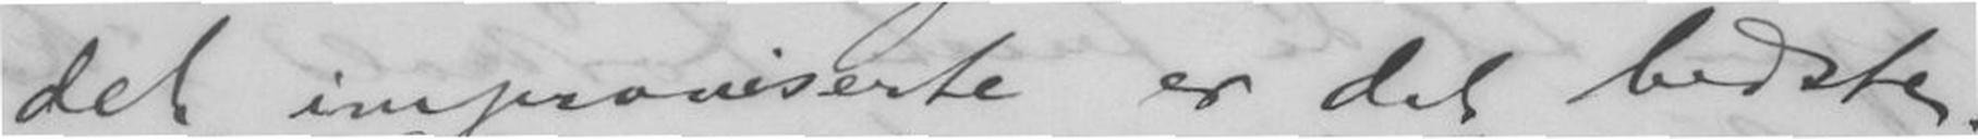det improviserte er det bedste.
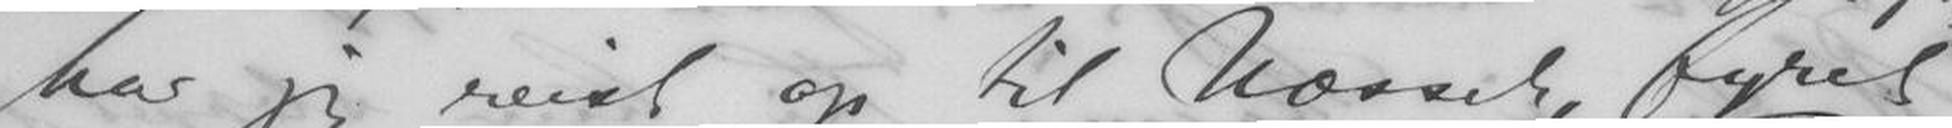har jeg reist op til Næsset, fyret
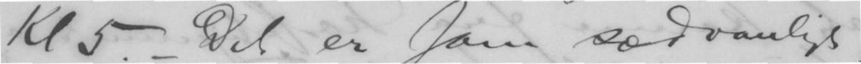Kl 5. - Det er som sædvanligt,
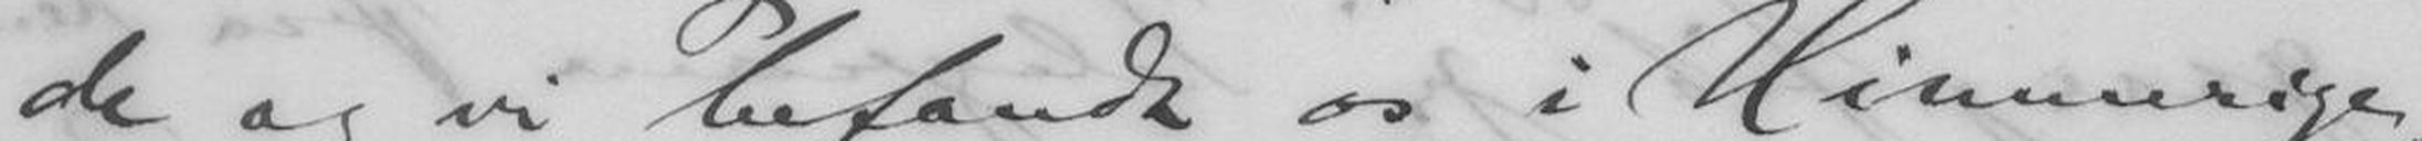de og vi befandt os i Himmerige,
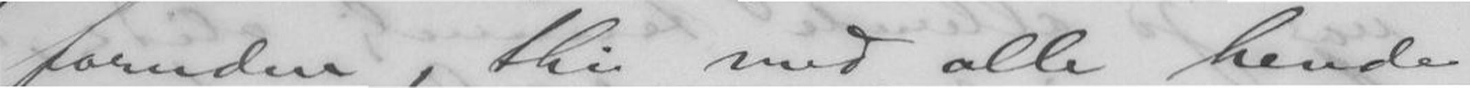foruden, thi med alle hendes
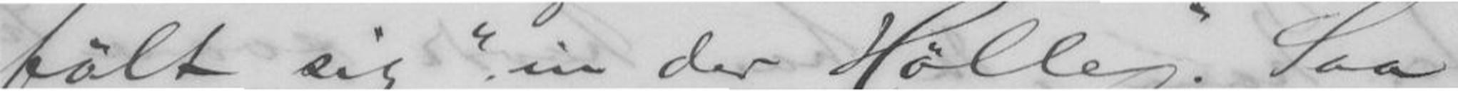følt sig "in der Hölle". Saa
